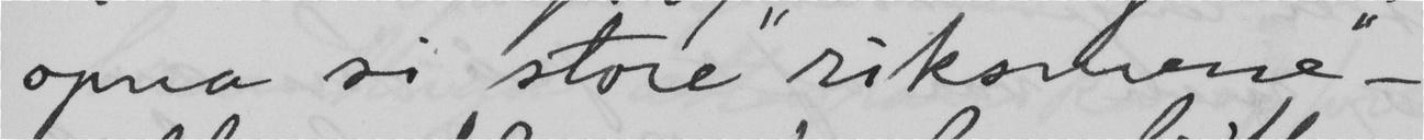opna si store "riksmesse" –
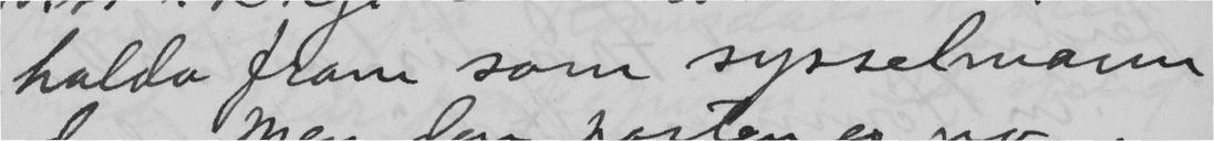halde fram som sysselmann
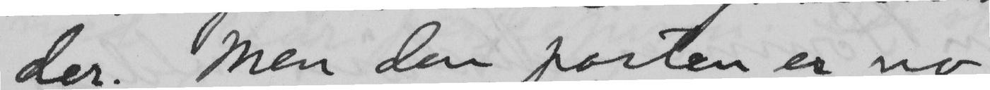der. Men den posten er no
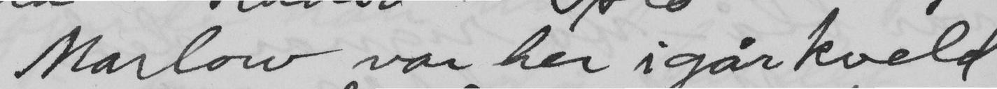Marlow var her igår kveld
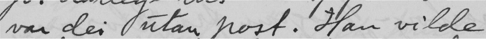var dei utan post. Han vilde
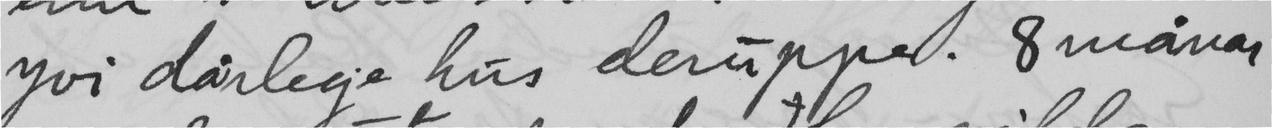yvi dårlege hus deruppe. 8 månar
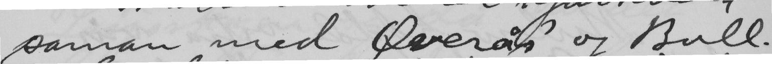saman med Øverås og Bull.
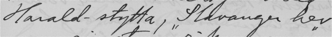Harald-stytta, Stavanger hev
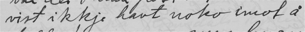vist ikkje havt noko imot å
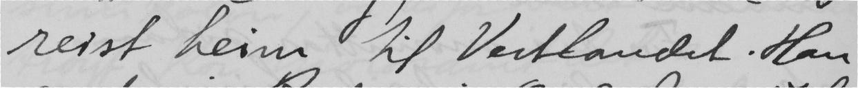reist heim til Vestlandet. Han
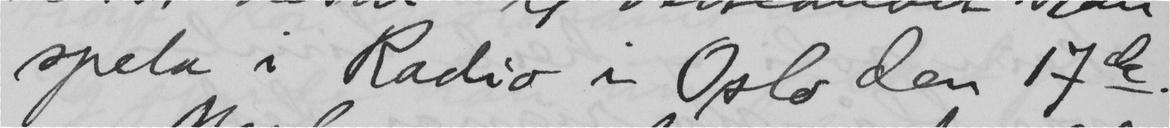spela i Radio i Oslo den 17de.
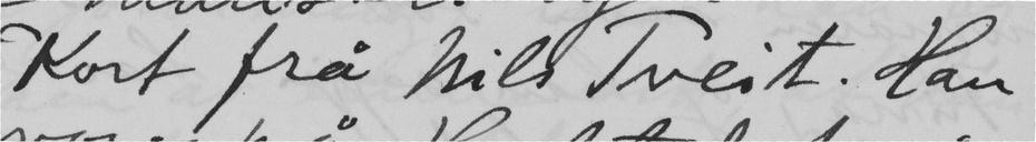Kort frå Nils Tveit. Han
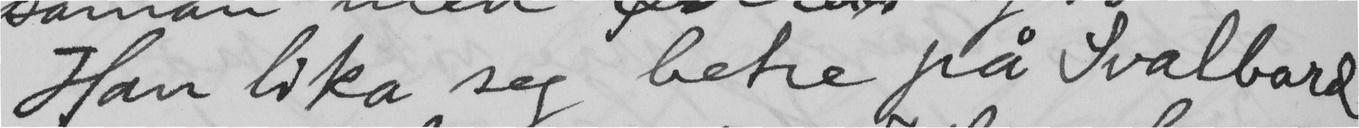Han lika seg betre på Svalbard
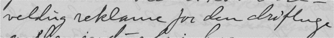veldug reklame for den driftuge
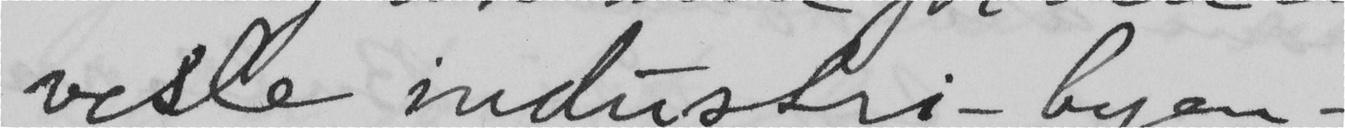vesle industri-byen –
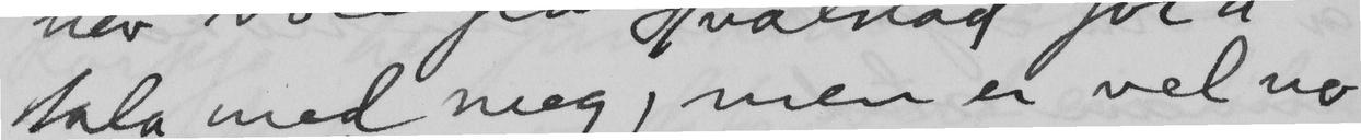tala med meg, men er vel no
